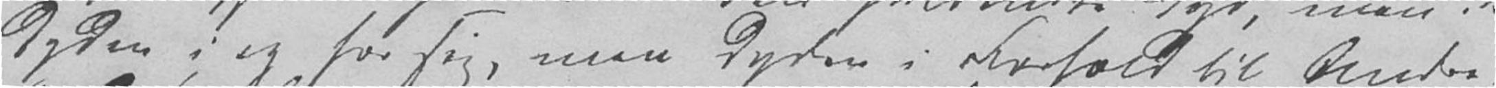Dyden i og for sig, men Dyden i Forhold til Andre.
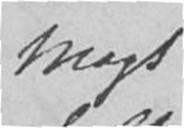Magt
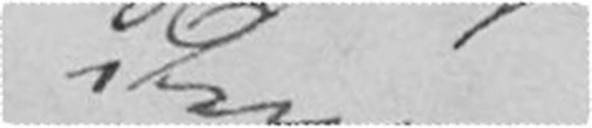Denne
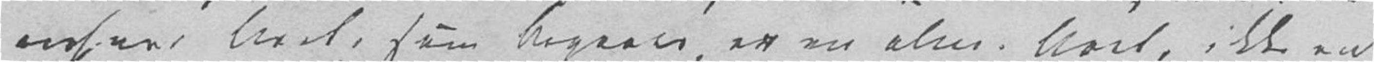enhver Uret, som begaaes, er en alm. Uret, ikke en
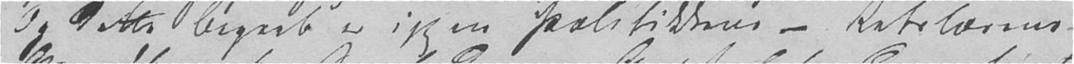I dette Begreb er igjen Politikkens – Retslærens –
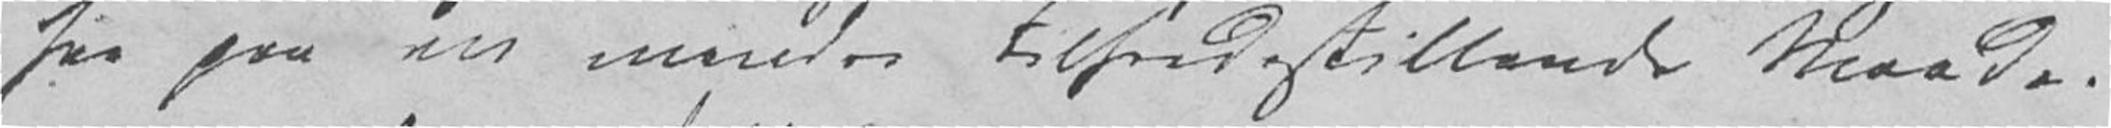see paa en mindre tilfredsstillende Maade.
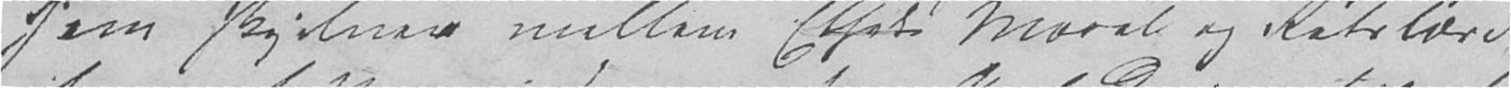som skjelner mellem Ethik Moral og Retslære
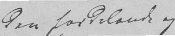den fordelende og
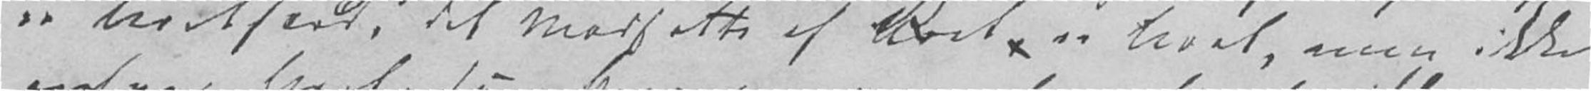er Uretfærd, det Modsatte af Ret er Uret, men ikke
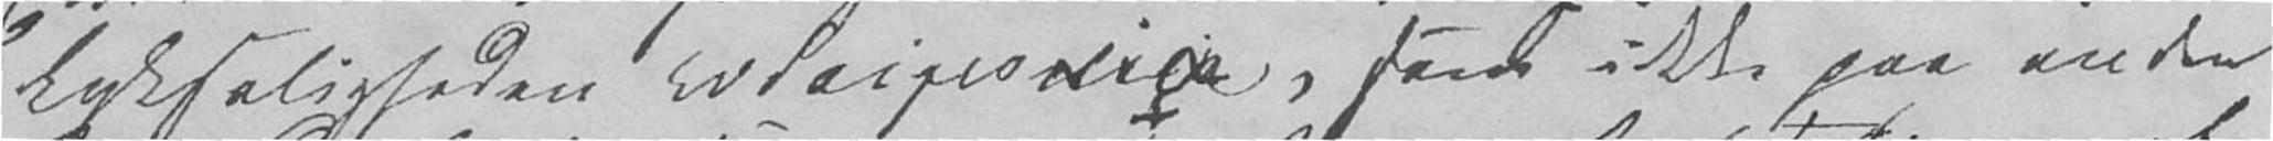Lyksaligheden øsoprnes, som ikke paa anden
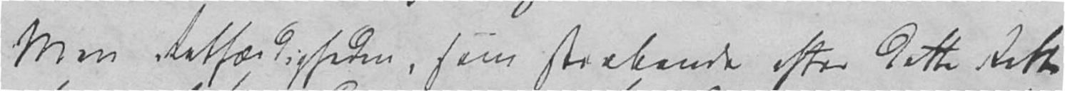Men Retfærdigheden, som stræbende efter dette Rette
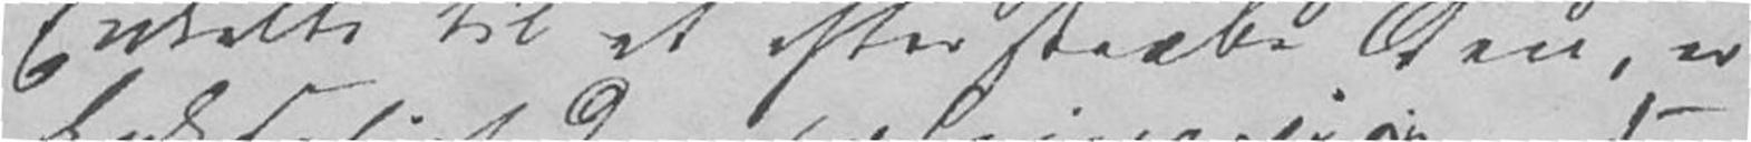Enkelte til at efterstræbe den, er
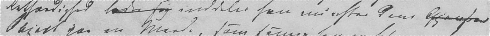Retfærdighed lader sig inddeler han nu efter dens Gjenstand
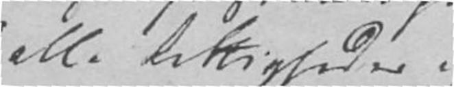alle Rettigheder og
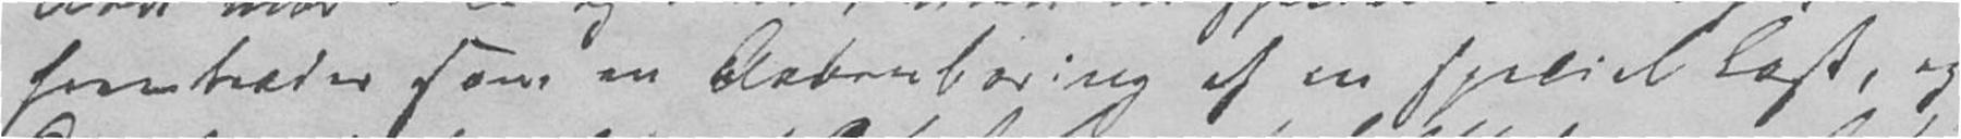fremtræder som en Aabenbaring af en speciel Last, og
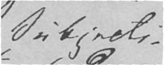Subjecti-
